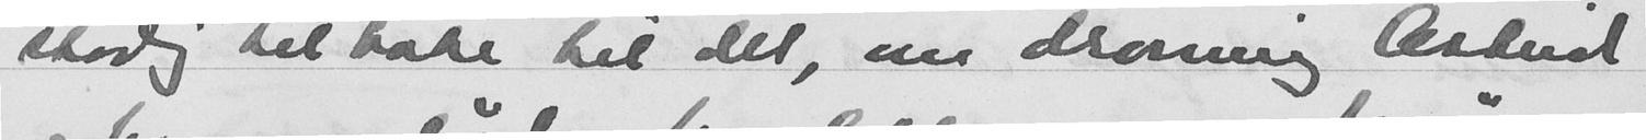stadig til bake til det, om dronnig Astrid
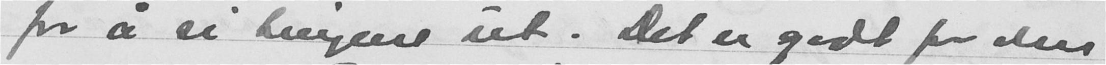for å si tingene ut. Det er godt for den
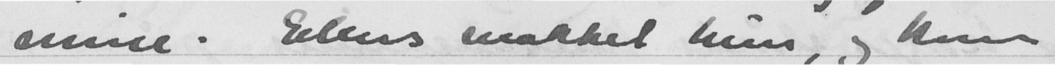mine. Ellers snakket hun, og kom
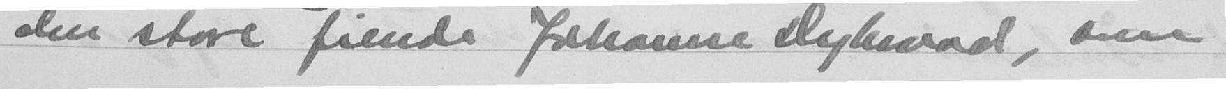den store fiende Johanne Dybwad, som
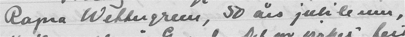Ragna Wettergreen, 50 års jubileum,
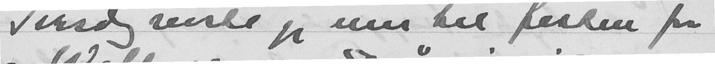Tirsdag reiste jeg inn til festen for
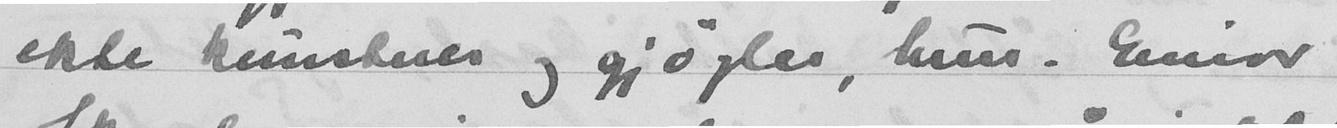ekte kunstnes og gjøgler, hun. Einar
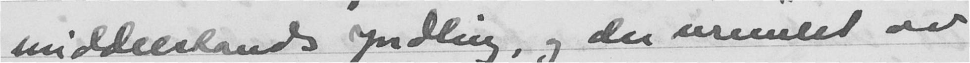middelstands yndling, og der vrimlet av
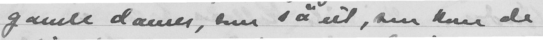gamle damer, som så ut, som kom de
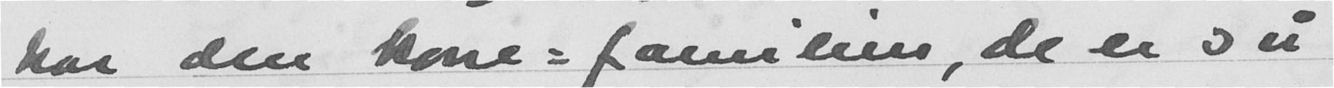har den kone-familien, de er så
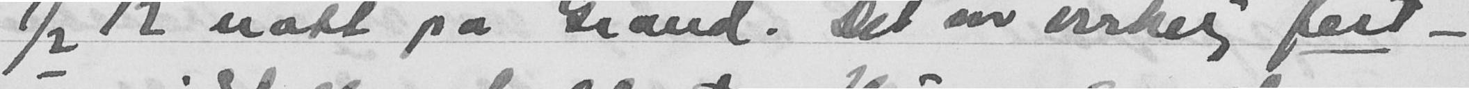1/2 12 natt på Grand. Det var virkelig fest -
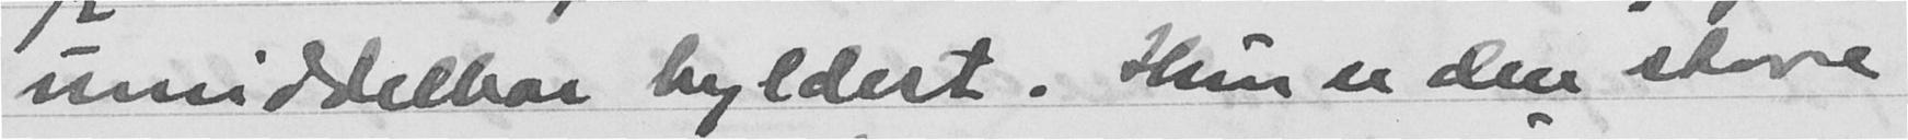umiddelbar hyldest. Hun er den store
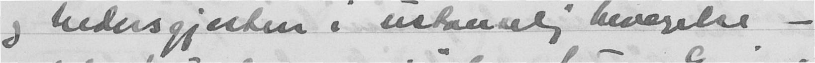og hedersgjesten i ustanselig bevegelse -
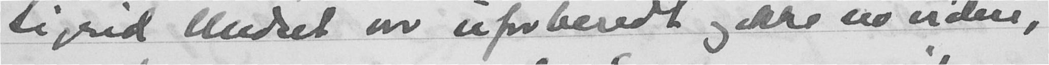Sigrid Undset var uforberedt og ikke no videre,
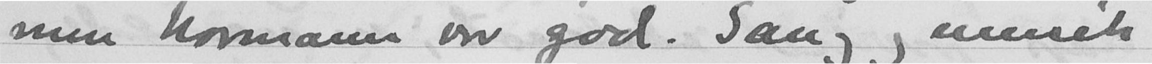men Normann var god. Sang og musik
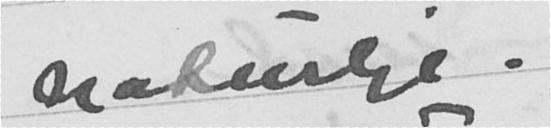naturlige.
